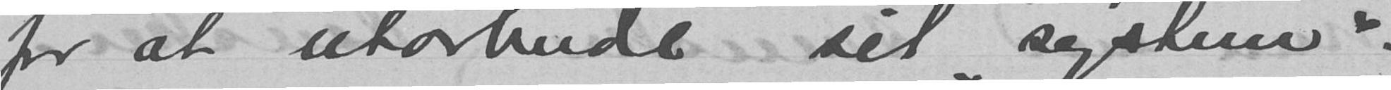for at utarbeide sit "system".
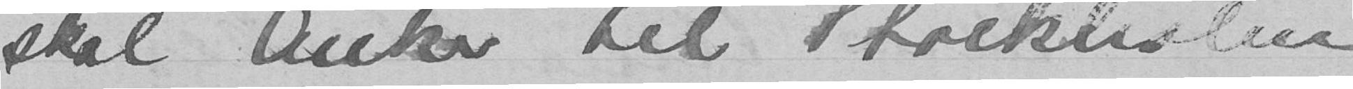skal Anker til Stockholm
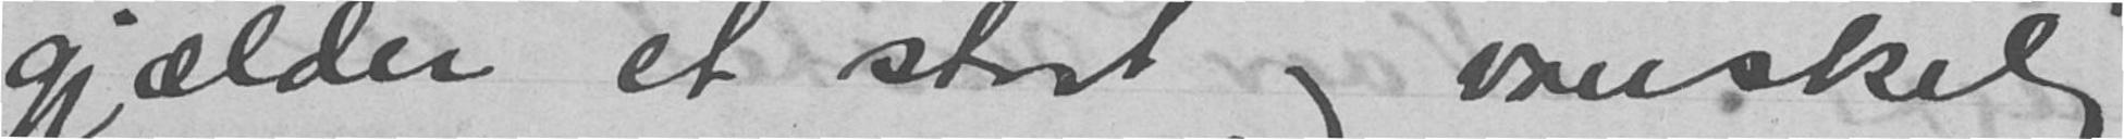gjælder et stort og vanskelig
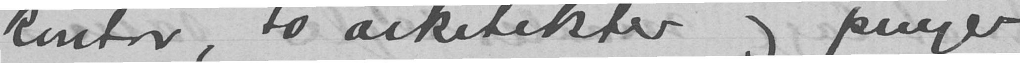kontor, to arkitekter og penger
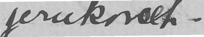jernkorset -
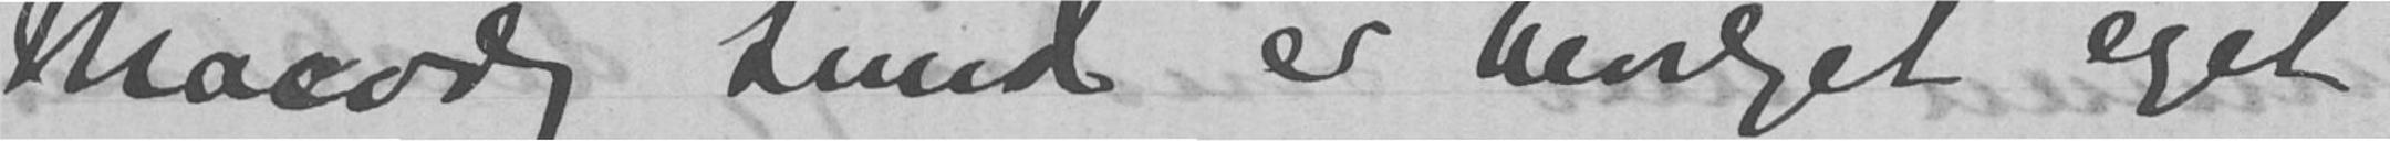Macody Lund er bevilget eget
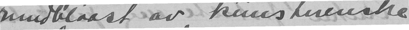rundblaast av "kunstneriske"
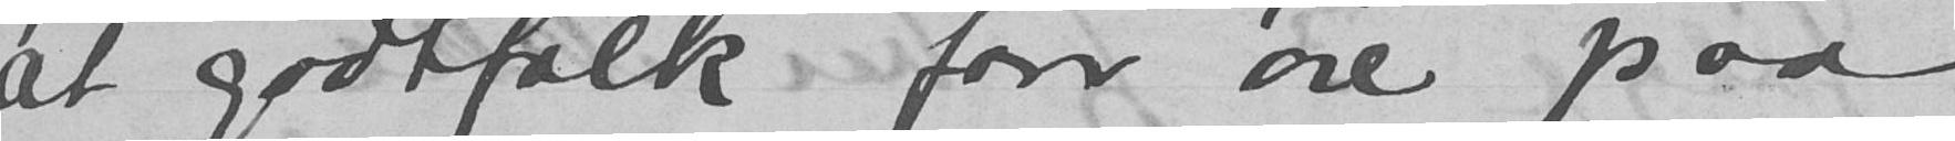at godtfolk faar øie paa
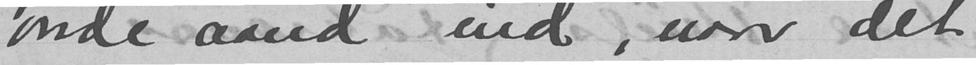onde aand ind, naar det
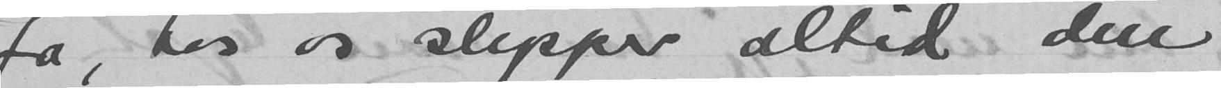Ja, hos os slipper altid den
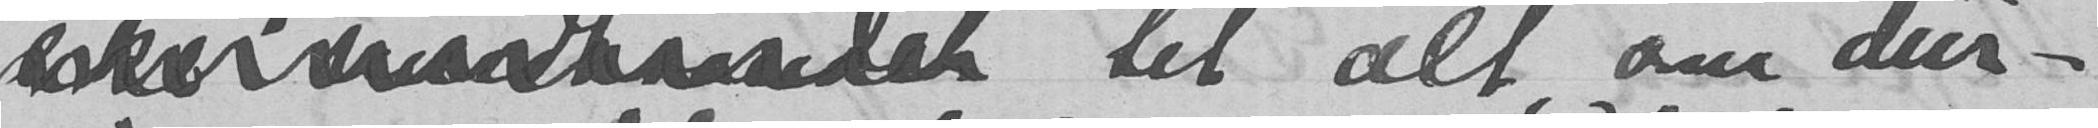til alt, som dur
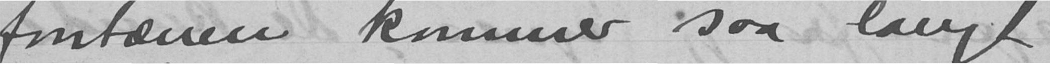fontænen kommer saa langt
